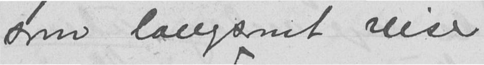som langsomt reiser
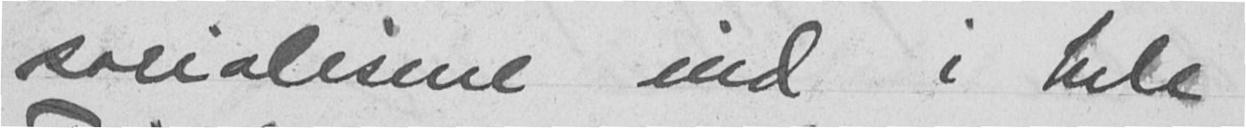socialisme ind i hele
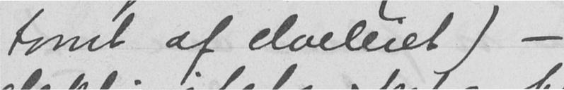tomt af elveleiet) -
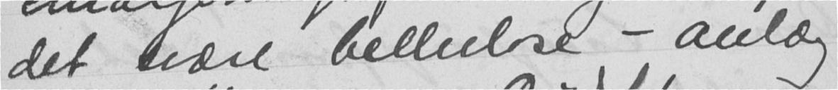det svære Cellulose-anlæg
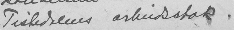Tistedalens arbeidsstok.
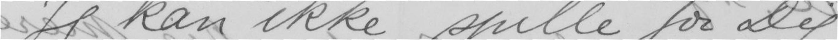Jeg kan ikke spille for Dig
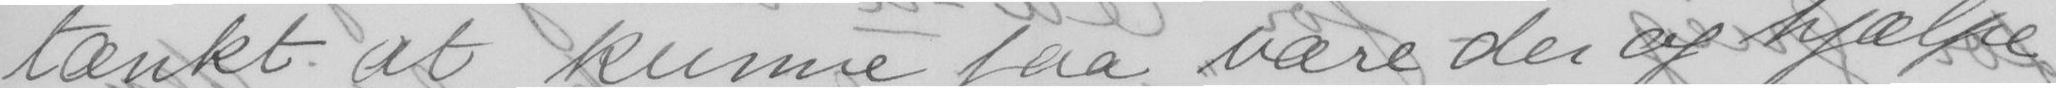tænkt at kunne faa være der og hjælpe
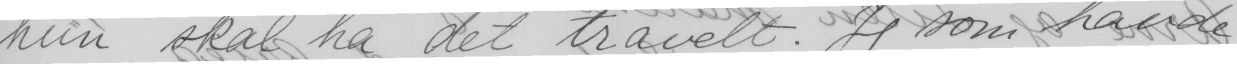hun skal ha det travelt. Jeg som havde
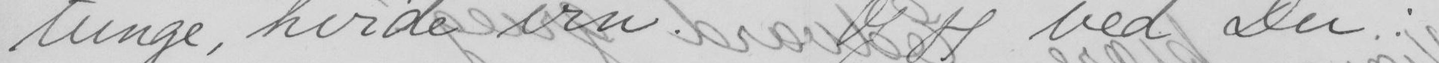tunge hvite vin. Og jeg ved Du:
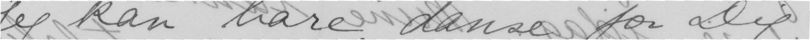Jeg kan bare danse for Dig
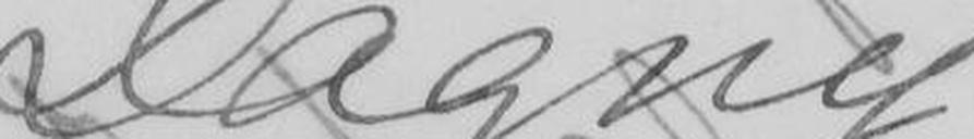Dagny
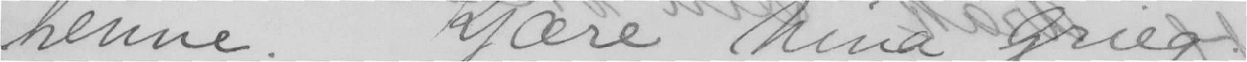henne.Kjære Nina Grieg!
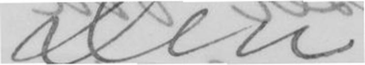Din
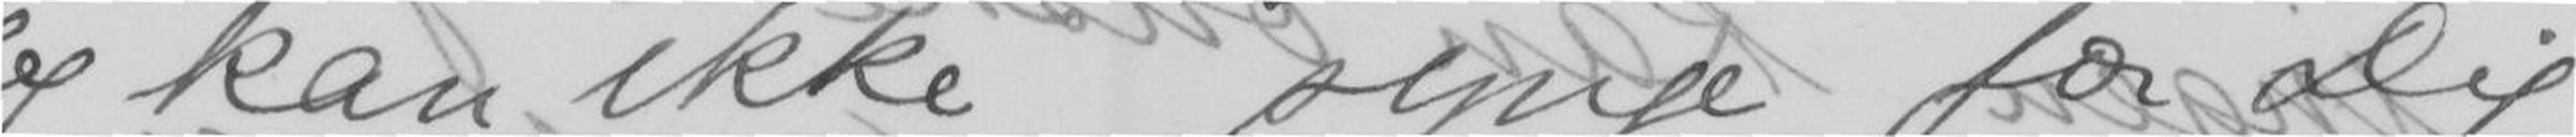Jeg kan ikke synge for Dig
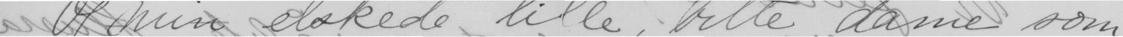Og Min elskede lille, bitte dame som
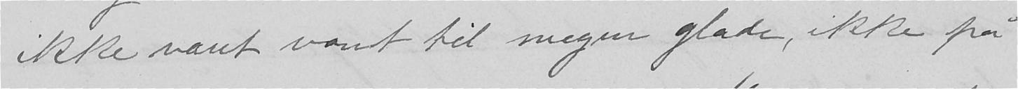ikke vært vant til megen glæde, ikke på
